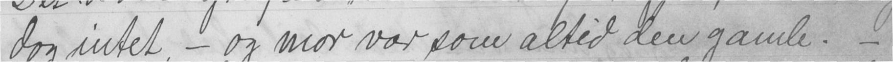dog intet, - og mor var som altid den gamle. -
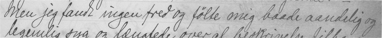Men jeg fandt ingen fred og følte mig baade aandelig og
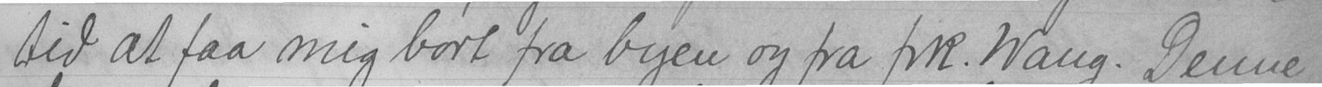tid at faa mig bort fra byen og fra frk. Wang. Denne
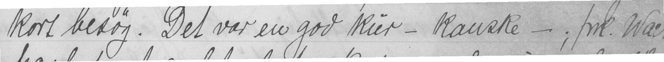kort besøg. Det var en god kur - kanske - ; frk. Wa
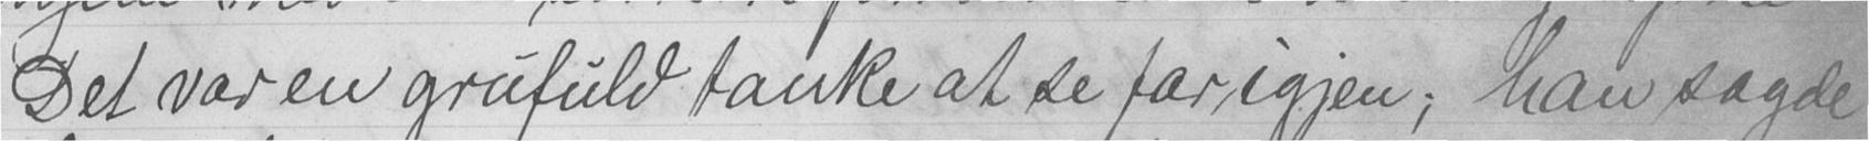Det var en grufuld tanke at se far igjen; han sagde
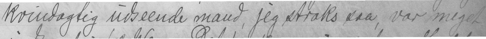kvindagtig udseende mand, jeg straks saa, var meget
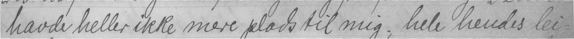havde heller ikke mere plads til mig; hele hendes lei-
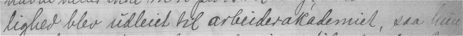lighed blev udleiet til arbeiderakademiet, saa hun
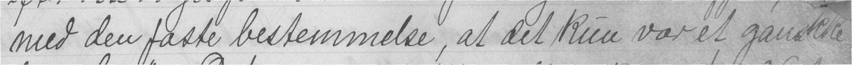med den faste bestemmelse, at det kun var et ganskke
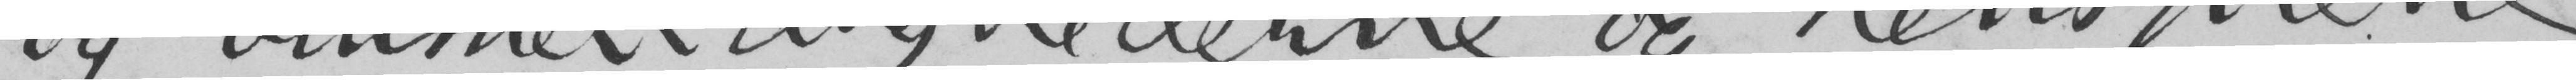og omstændighederne og hensynene
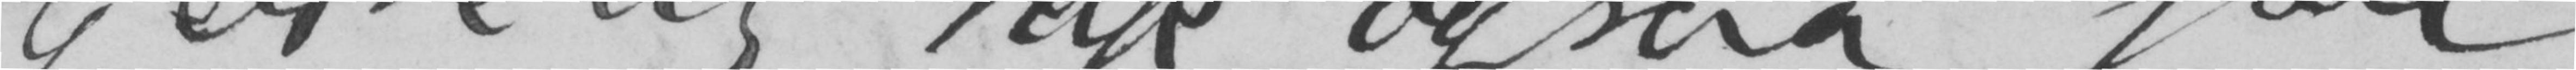Hjertelig tak ogsaa for
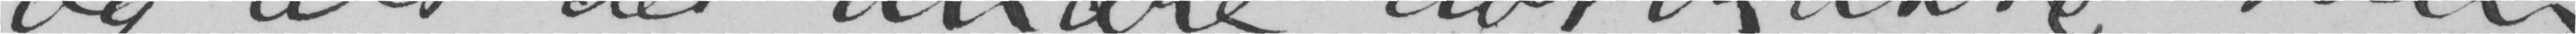og alt det andre abstrakte, som
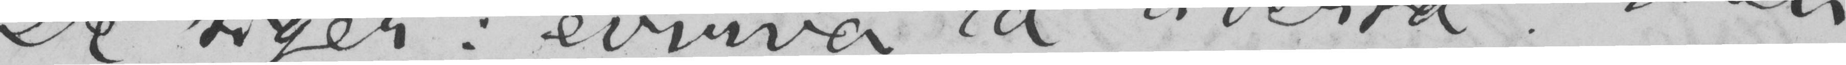De siger: evviva la libertà! Men
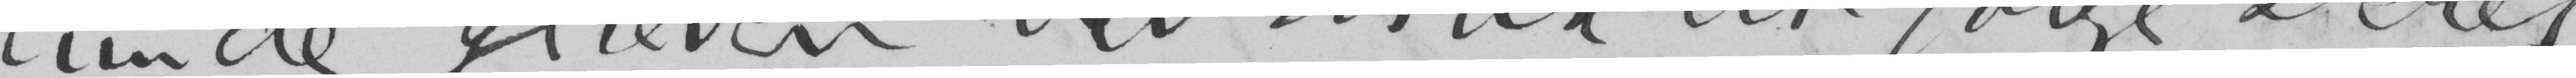lunde glæden ved strax at følge Deres
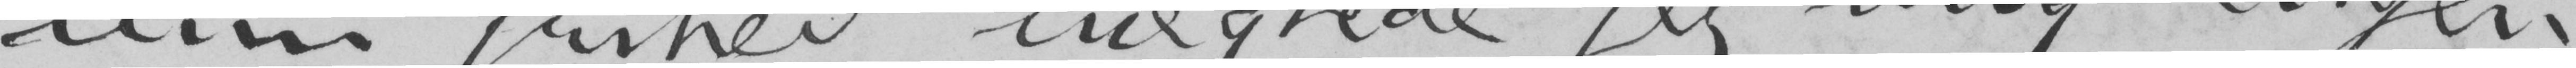min frihed, nægtede jeg mig ingen-
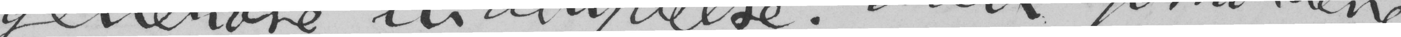generøse indbydelse. Men forholdene
